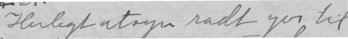Herlegt utsyn sadt yvi til
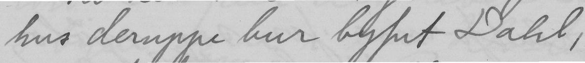hus deruppe bur byfut Dahl,
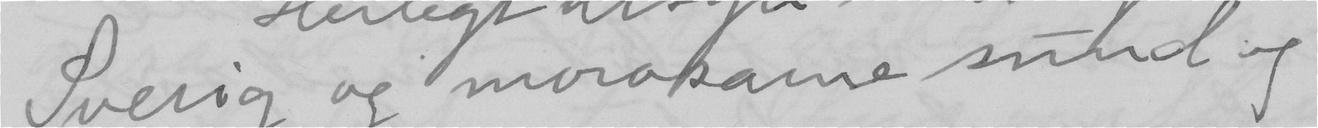Sverig og morosame sund og
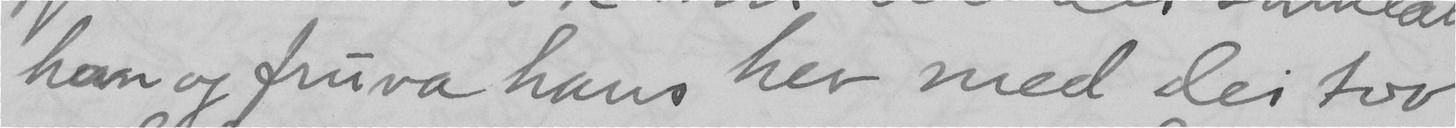han og fruva hans hev med dei tvo
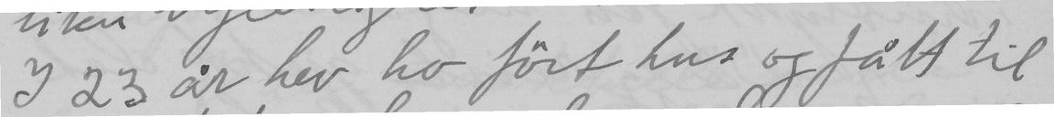I 23 år hev ho fört hus og fått til
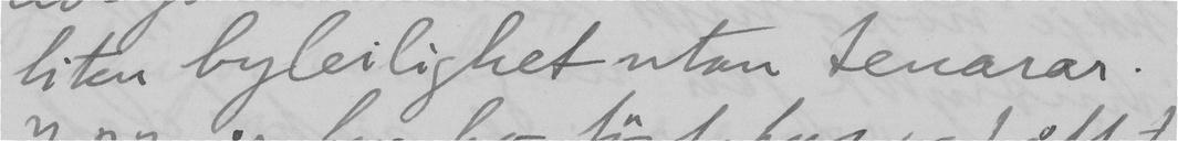liten byleilighet utan tenarar.
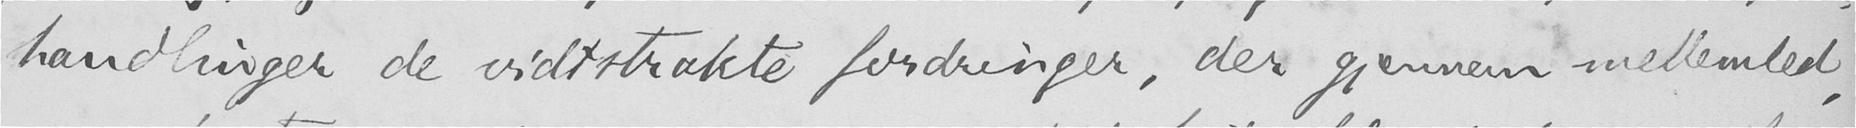handlinger de vidtstrakte fordringer, der gjennem mellemled,
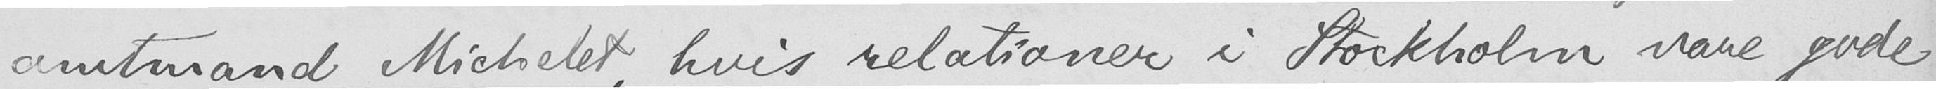amtmand Michelet, hvis relationer i Stockholm vare gode
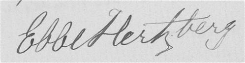Ebbe Hertzberg.
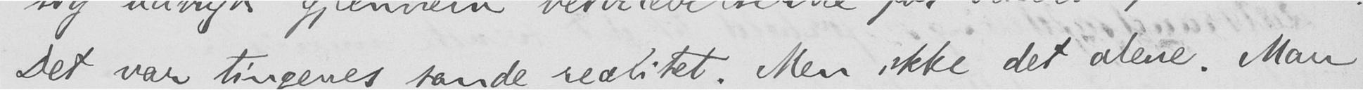Det var tingenes sande realitet. Men ikke det alene. Man
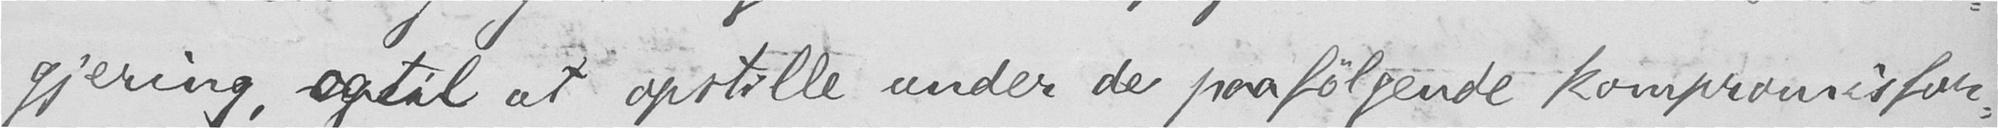gjering, og til at opstille under de paafølgende kompromisfor-
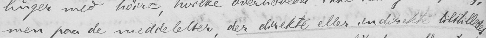men paa de meddelelser, der direkte eller indirekte tilstilledes
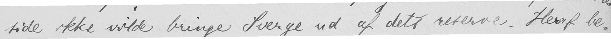side ikke vilde bringe Sverge ud af dets reserve. Heraf be-
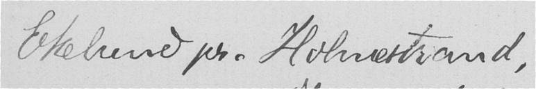Ekelund pr. Holmestrand,
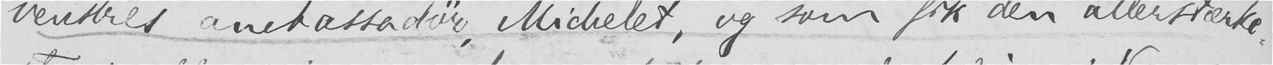venstres ambassadør, Michelet, og som fik den allerstærke-
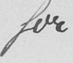for
